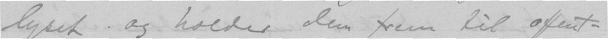lyset og holder dem frem til offent-
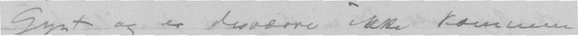Gynt og er desværre ikke kommen
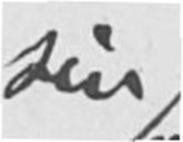sin
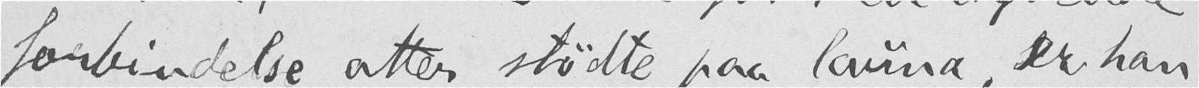forbindelse atter stødte paa launa, er han
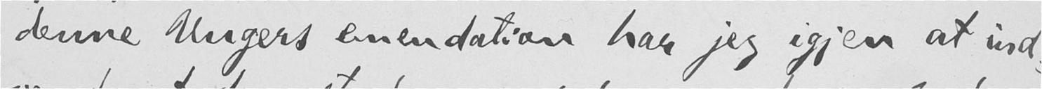denne Ungers emendation har jeg igjen at ind-
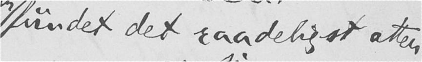fundet det raadeligst atter
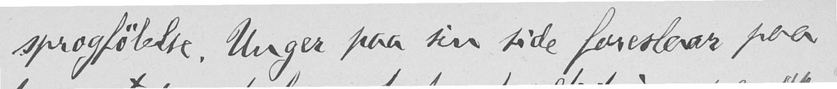sprogfølelse. Unger paa sin side foreslaar paa
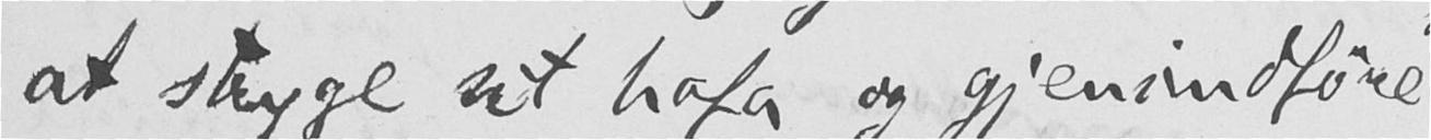at stryge sit hafa og gjenindføre
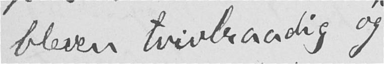bleven tvivlraadig og
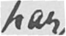har
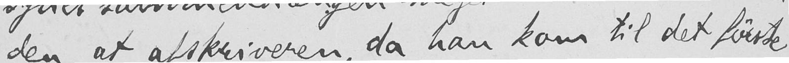den, at afskriveren, da han kom til det første
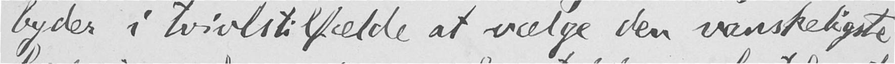byder i tvivlstilfælde at vælge den vanskeligste
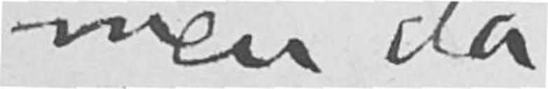men da
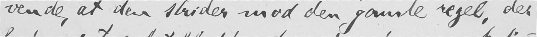vende, at den strider mod den gamle regel, der
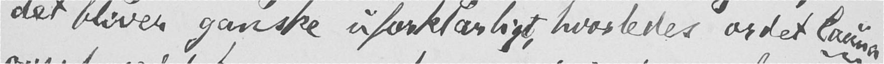det bliver ganske uforklarligt, hvorledes ordet launa
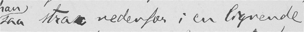saa strax nedenfor i en lignende
